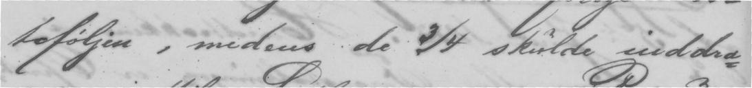teføljen, medens de ¾ skulde inddra-
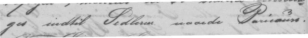ges indtil Sedlerne naaede Paricours.
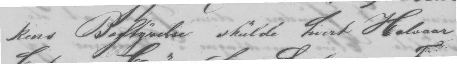kens Bestyrelse skulde hvert Halvaar
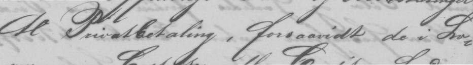Al Privatbetaling, forsaavidt de i Lo-
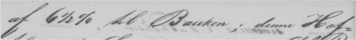af 6½ % til Banken; denne Hæf-
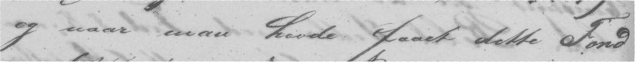og naar man havde faaet dette Fond
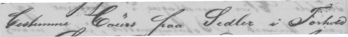bestemme Cours paa Sedler i Forhold
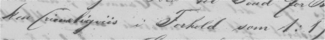ken (vaneligviis i Forhold som 1:1)
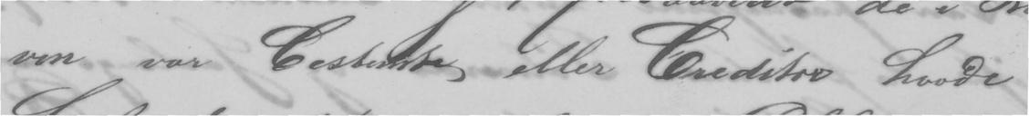ven var bestemte eller Creditor havde
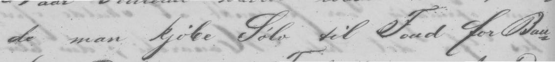de man kjøbe Sølv til Fond for Ban-
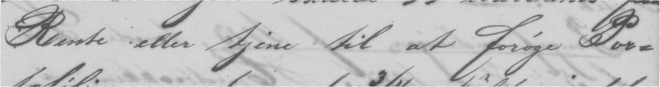Rente eller tjene til at forøge Por-
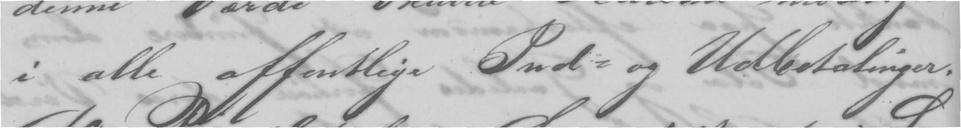i alle offentlige Ind- og Udbetalinger.
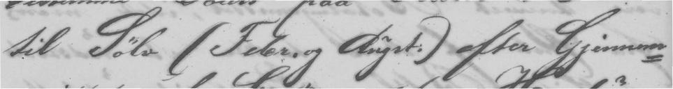til Sølv (Febr. og Augst.) efter Gjennom-
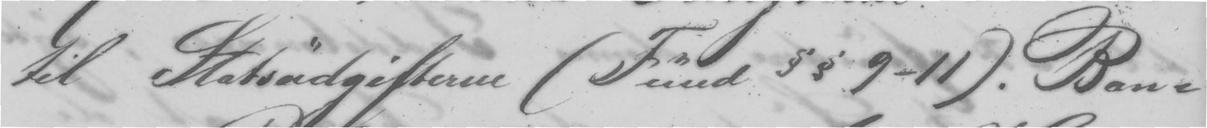til Statsudgifterne (Fund §§ 9-11). Ban-
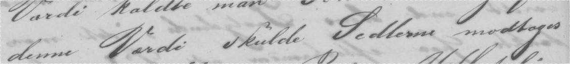denne Værdi skulde Sedlerne modtages
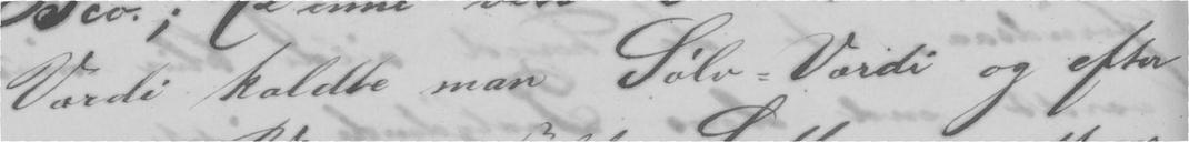Værdi kaldte man Sølv-Værdi og efter
